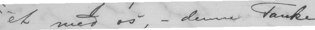et med os, - denne Tanke
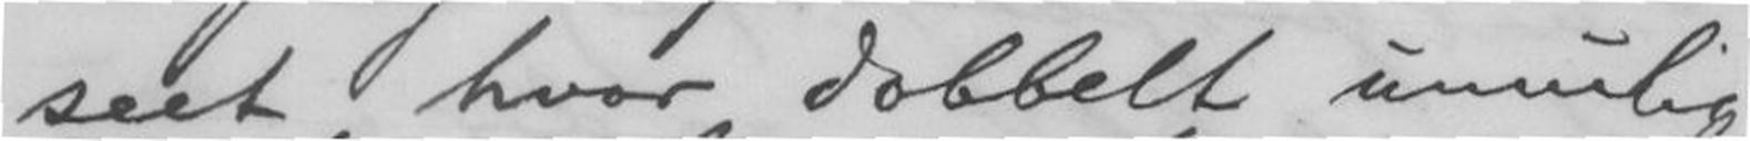seet, hvor dobbelt umulig
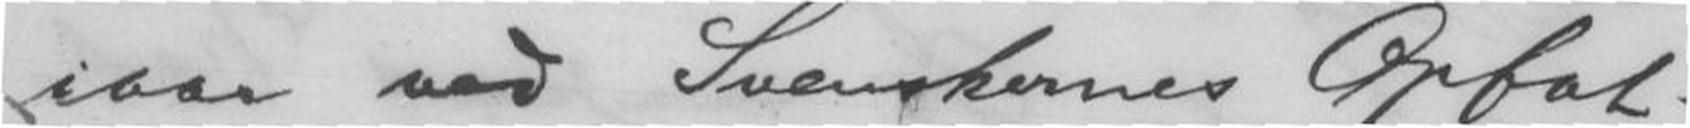iaar ved Svenskernes Opfat-
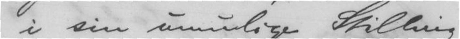i sin umulige Stilling
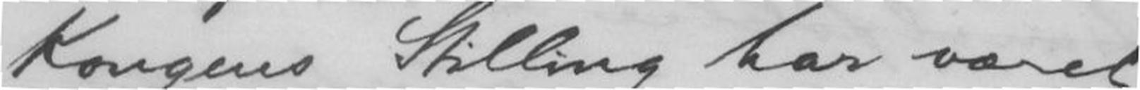Kongens Stilling har været
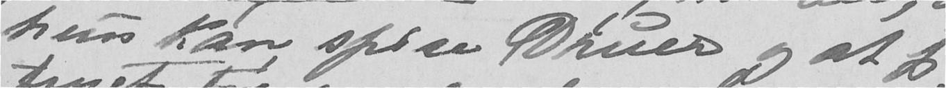hun kan spise Druer og at jeg
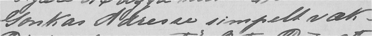Gonkas Adresse simpelt væk -
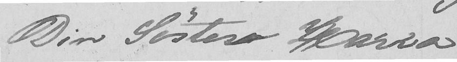Din Søster Harra
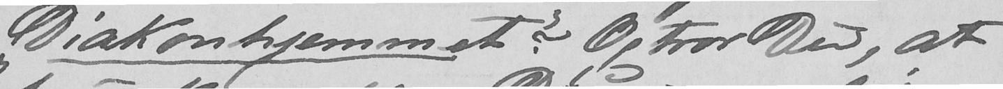Diakonhjemmet? Og tror Du, at
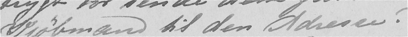Kjøbmand til den Adresse?
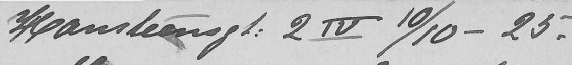Hansteensgt. 2 IV 10/10 - 25.
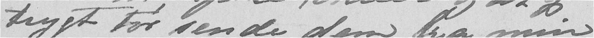trygt tør sende dem fra min
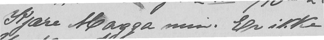Kjære Magga min. Er ikke
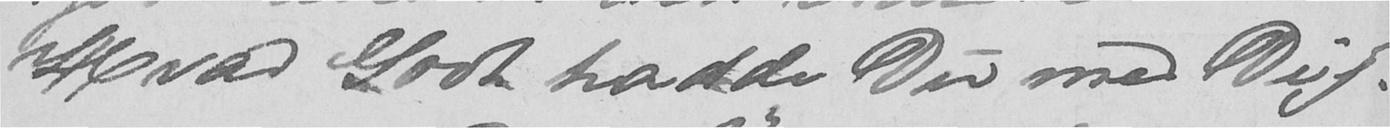Hvad Godt hadde Du med Dig?
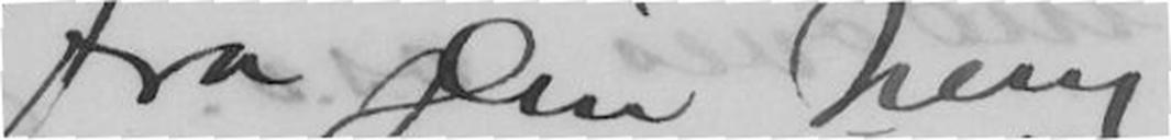fra Din heng.
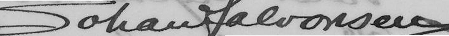Johan Halvorsen.
